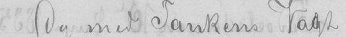Og med Tankens Vagt
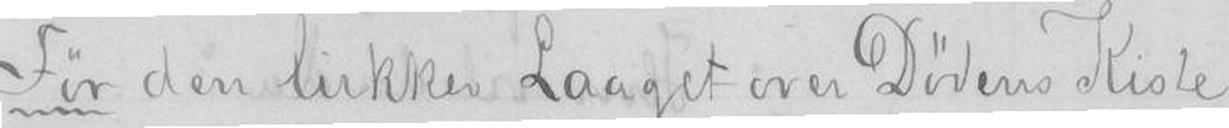Før den lukker laaget over Dødens Kiste
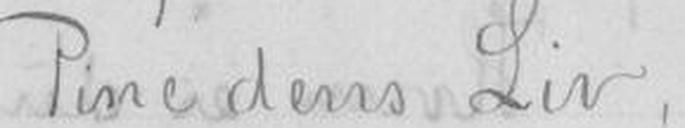Pine dens Liv,
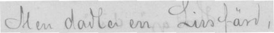Men dadler en Livsfärd,
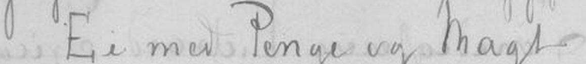Ei med Penge og Magt
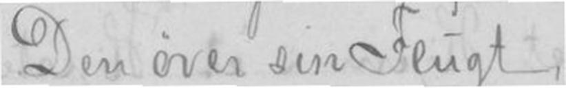Den øver sin Flugt.
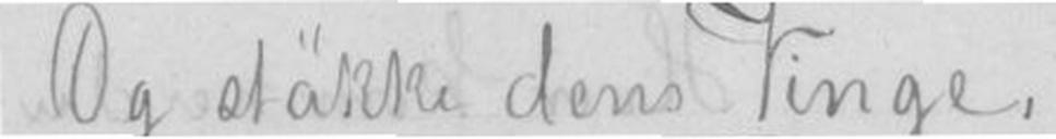Og stäkke dens Vinge,
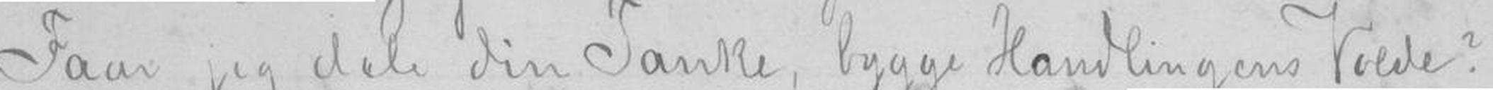Faar jeg dele din Tanke, bygge Handlingens Volde?
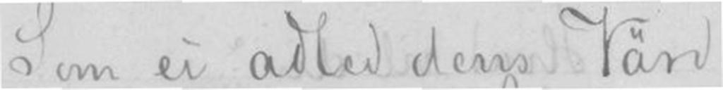Som ei adled dens Värd
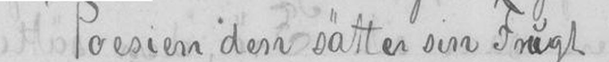Poesien den sätter sin Frugt
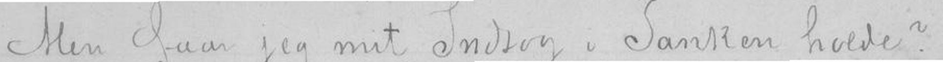Men faar jeg mit Indtog i Tanken holde?
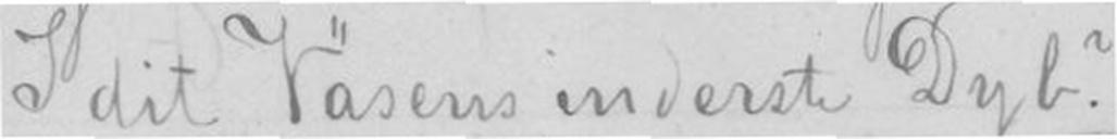I dit Väsens inderste Dyb?
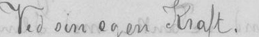Ved sin egen Kraft.
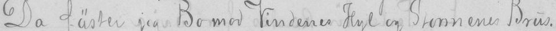Da fäster jeg Bo mod Vindenes Hyl og Stormenes Brus
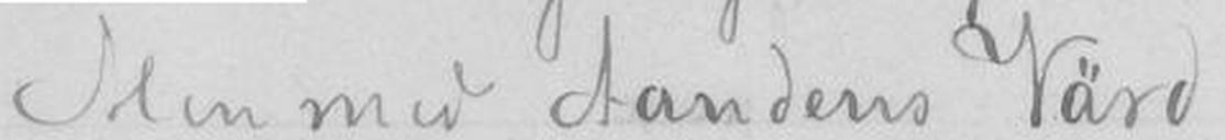Iden med Aandens Värd
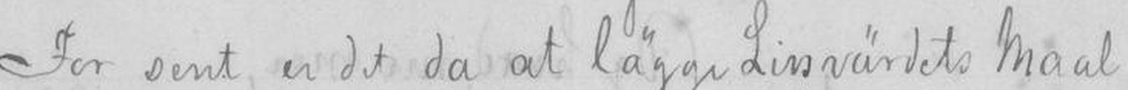For sent er det da at lägge Livsvärdets Maal
